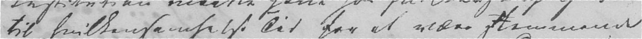til hvilkensomhelst Tid for at være stemmende
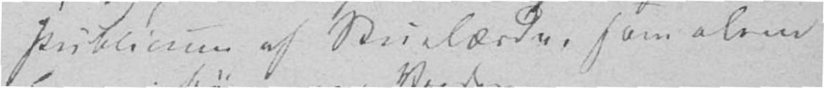Publicum af Skuelærde, som alene
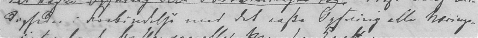digheder i Forbindelse med det raske Opsving alle Nærings-
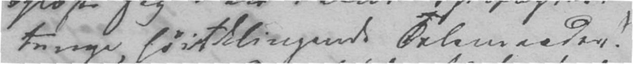tunge, høitklingende Talemaader.
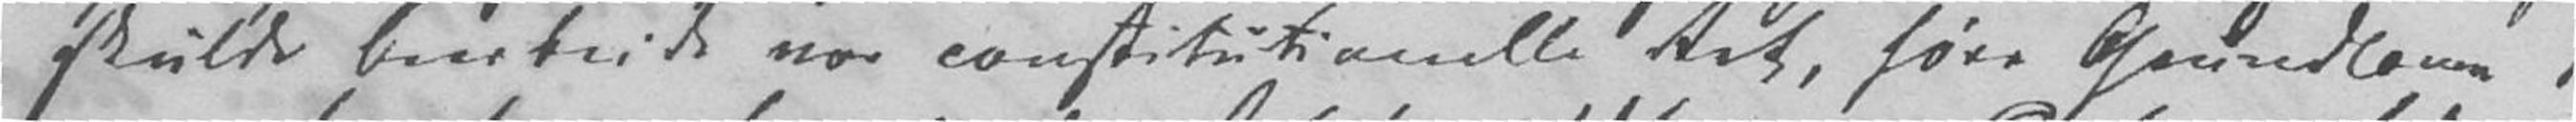skulde bearbeide vor constitutionelle Ret, føre Grundloven
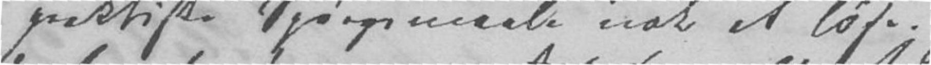praktiske Spørgsmaale nok at løse.
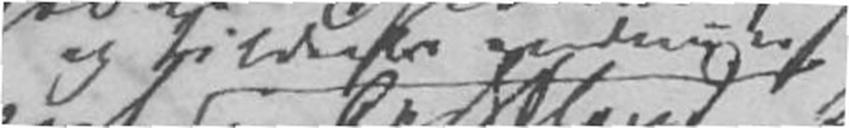og tildeels endnu er
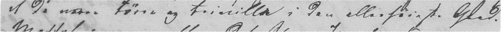at de vare tørre og trivielle i den allerhøieste Grad.
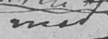med
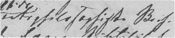retsphilosophiske Skrif-
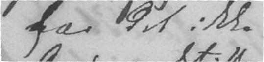har det ikke
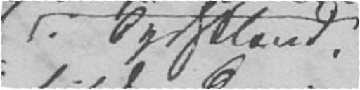i Tyskland,
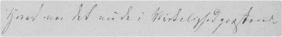Hvad var det nu de i Virkelighed præsterede
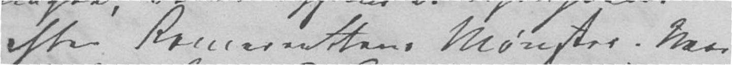efter Romerrettens Mønster. Naar
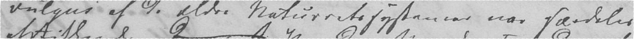vulgus af de ældre Naturretssystemer var særdeles
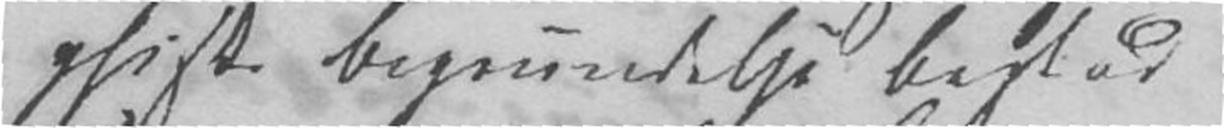phiske Begrundelse bestod
